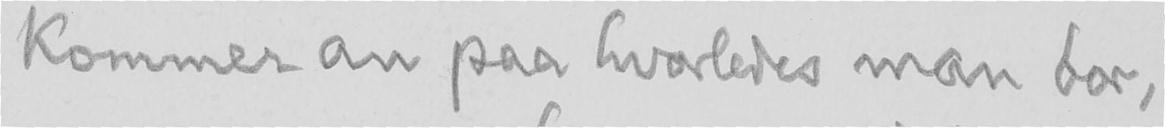kommer an paa hvorledes man bor,
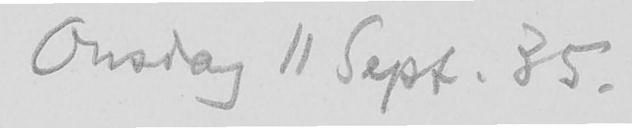Onsdag 11 Sept. 35.
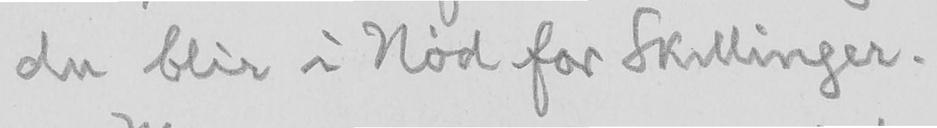du blir i Nød for Skillinger.
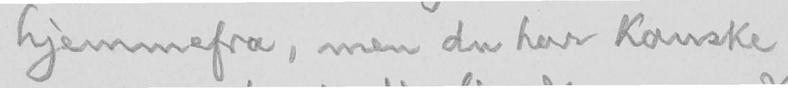hjemmefra, men du har kanske
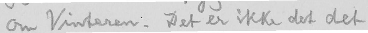om Vinteren. Det er ikke det det
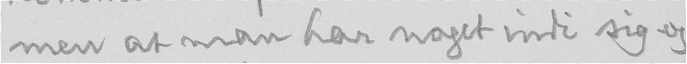men at man har noget indi sig og
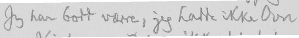Jeg har bodd værre, jeg hadde ikke Ovn
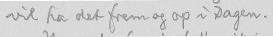vil ha det frem og op i Dagen.
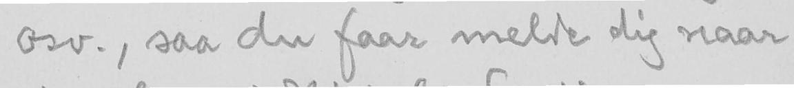osv., saa du faar melde dig naar
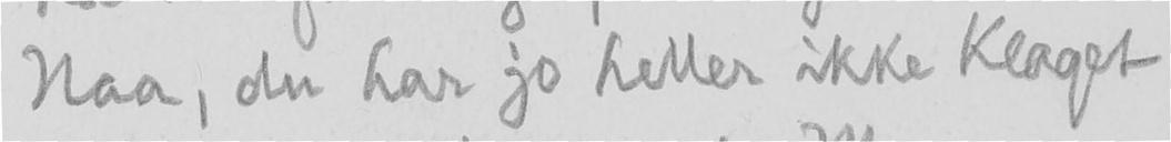Naa, du har jo heller ikke klaget
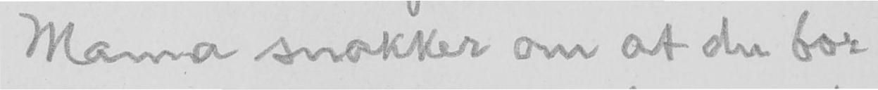Mama snakker om at du bor
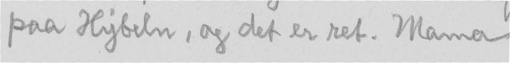paa Hybeln, og det er ret. Mama
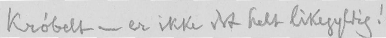krøbelt - er ikke det helt likegyldig!
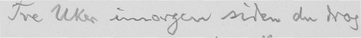Tre Uker imorgen siden du drog
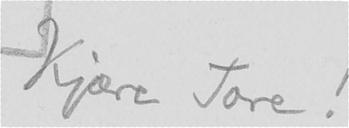Kjære Tore!
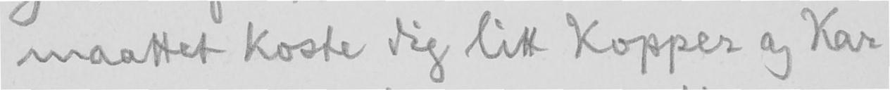maattet koste dig litt Kopper og Kar
